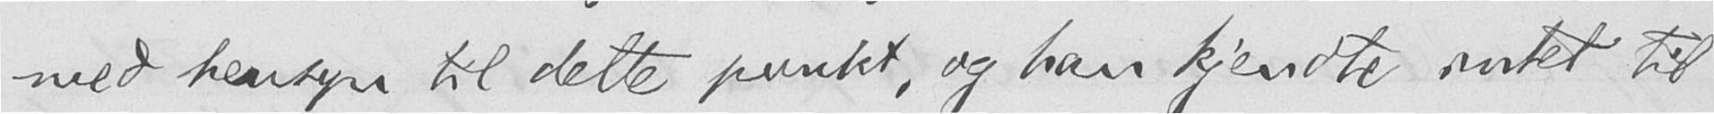med hensyn til dette punkt, og han kjendte intet til
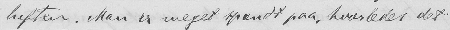luften. Man er meget spændt paa, hvorledes det
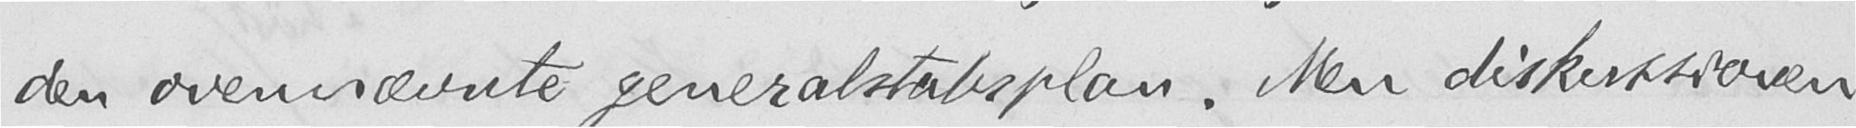den ovennævnte generalstabsplan. Men diskussionen
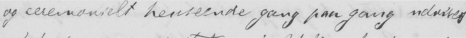og ceremonielt henseende gang paa gang udvises
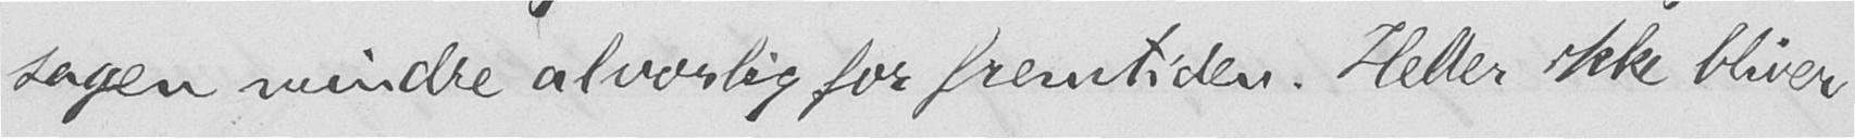sagen mindre alvorlig for fremtiden. Heller ikke bliver
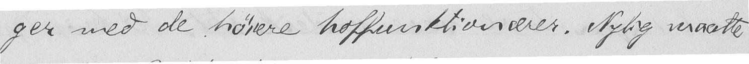ger med de høiere hoffunktionærer. Nylig maatte
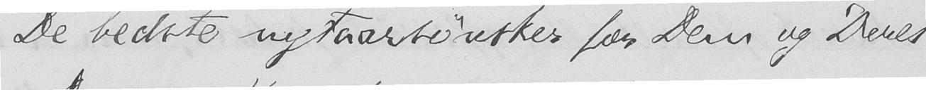De bedste nytaarsønsker for Dem og Deres
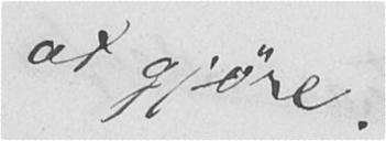at gjøre.
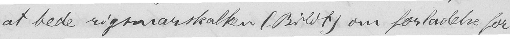at bede rigsmarskalken (Bildt) om forladelse for
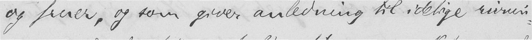og fruer, og som giver anledning til idelige rivnin-
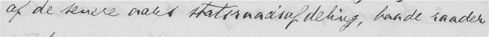af de senere aars statsraadsafdeling, baade raader
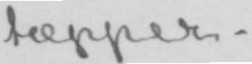tæpper.
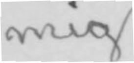mig
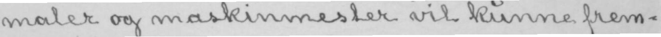maler og maskinmester vil kunne frem-
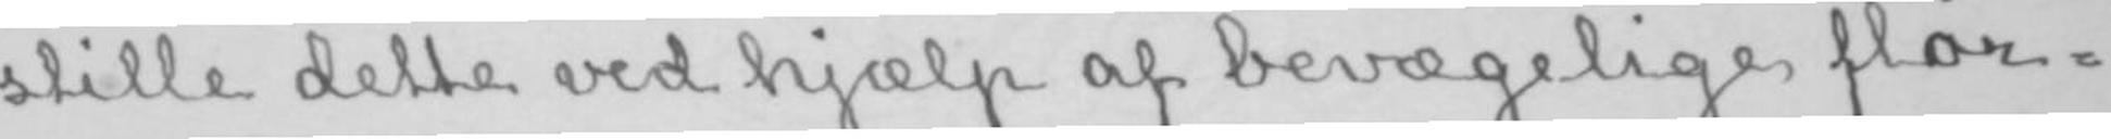stille dette ved hjælp af bevægelige flor-
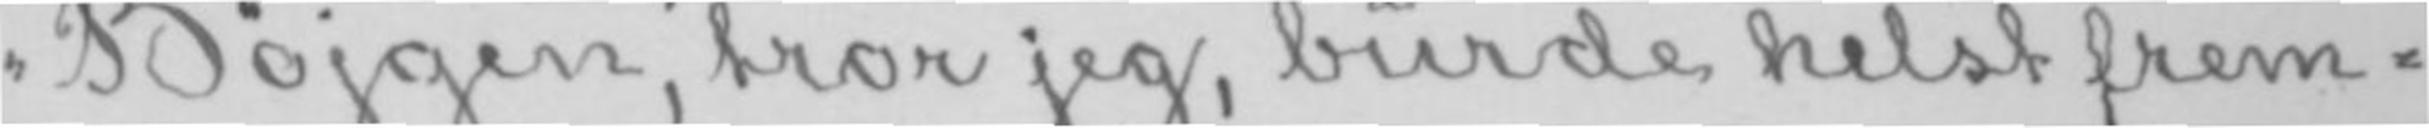«Bøjgen», tror jeg, burde helst frem-
